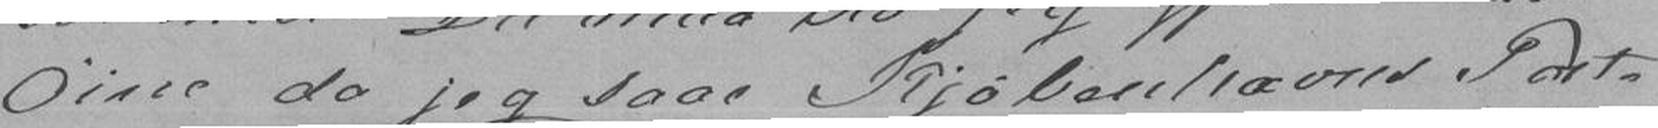Øine da jeg saae Kjøbenhavn Post-
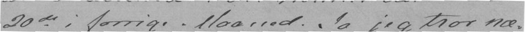20de i forrige Maaned. Jo jeg tror næ-
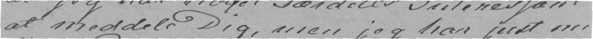at meddele Dig, men jeg har just nu
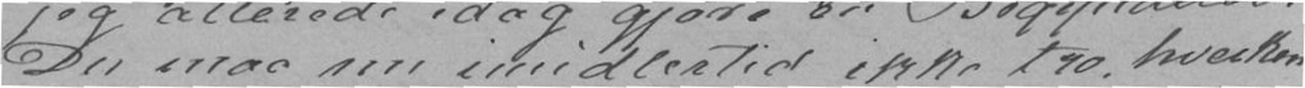Du maa nu imidlertid ikke tro hverken
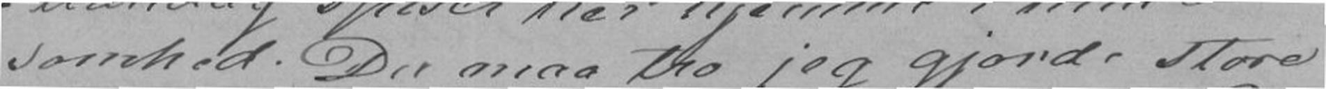somhed. Du maa tro jeg gjorde store
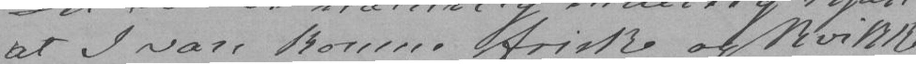at I vare komne friske og kvikke
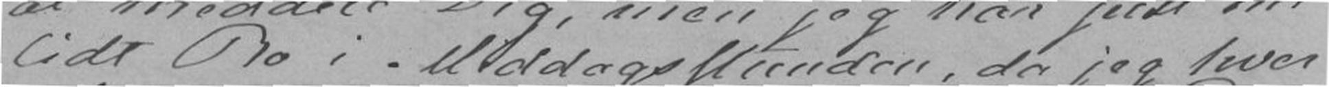lidt Ro i Middagsstunden, da jeg hver
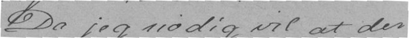Da jeg nødig vil at der
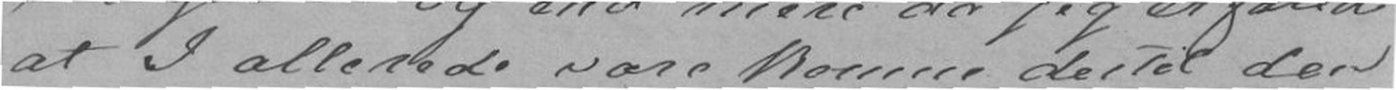at I allerede vare komne dertil den
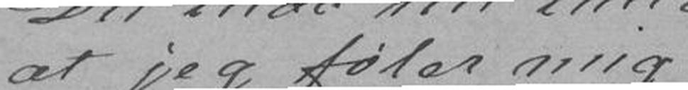at jeg føler mig
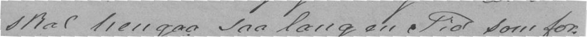skal hengaa saa lang en Tid som for-
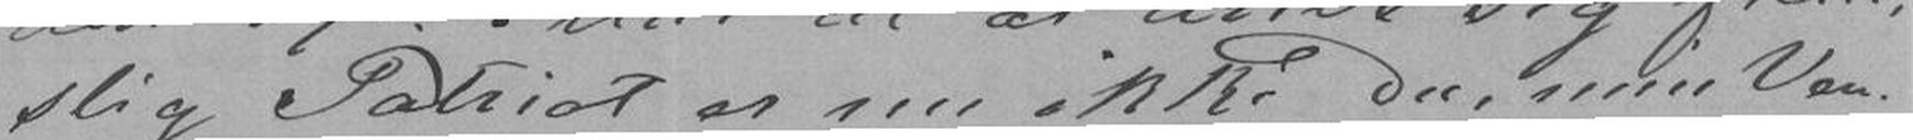slig Patriot er nu ikke Du, min Ven.
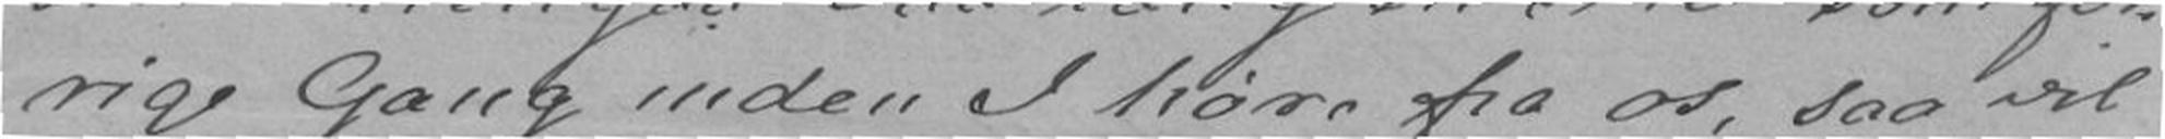rige Gang inden I høre fra os, saa vil
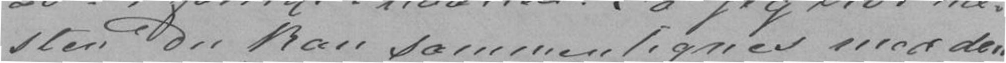sten Du kan sammenlignes med den
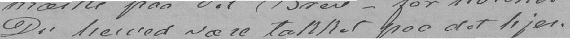Du herved være takket paa det hjer-
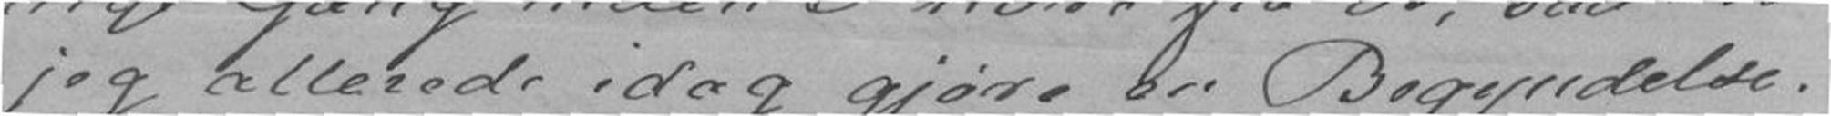jeg allerede idag gjøre en Begyndelse.
